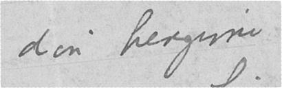din hengivne
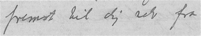fremst til dig selv fra
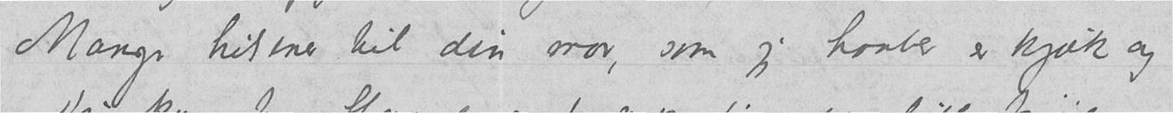Mange hilsener til din mor, som jeg haaber er kjæk og
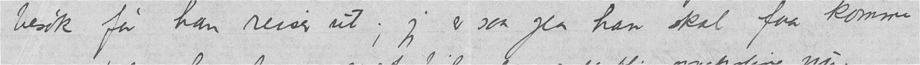besøk før han reiser ut; jeg er saa gla han skal faa komme
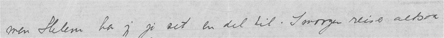men Helene har jeg jo set en del til. Imorgen reiser altsaa
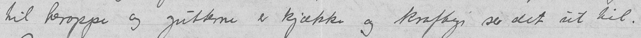til heroppe og gutterne er kjække og kraftige ser det ut til.
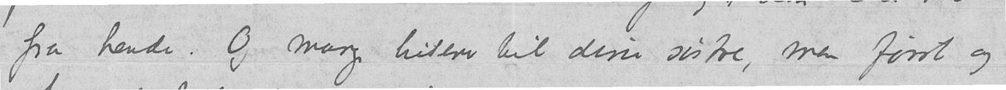fra hende. Og mange hilsener til dine søstre, men først og
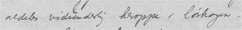aldeles vidunderlig heroppe i løvhagen.
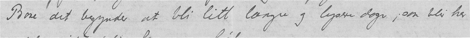Bare det begynder at bli litt længre og lysere dager, saa blir her
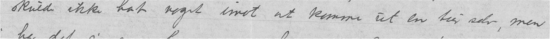Jeg skulde ikke hat noget imot at komme ut en tur selv, men
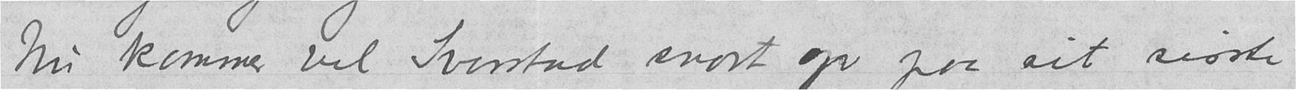Nu kommer vel Svarstad snart op paa sit sisste
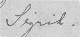Sigrid.
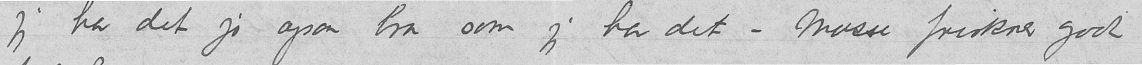jeg har det jo ogsaa bra som jeg har det – Mosse friskner godt
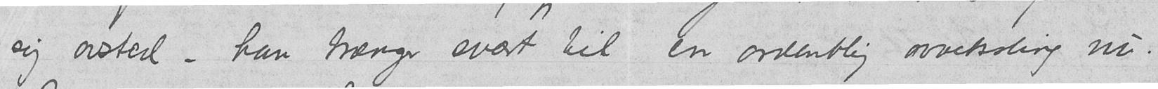sig avsted – han trænger svært til en ordentlig avveksling nu.
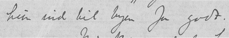hun ind til byen for godt.
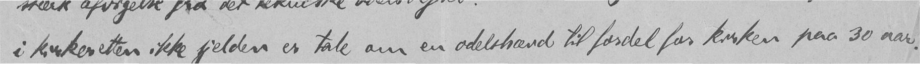i kirkeretten ikke sjelden er tale om en odelshævd til fordel for kirken paa 30 aar.
